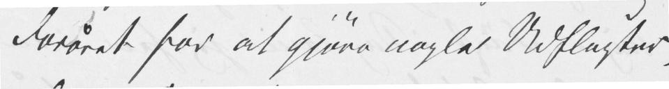Foråret for at gjøre nogle Udflugter
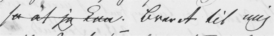sa at jeg kan. Brevet til mig
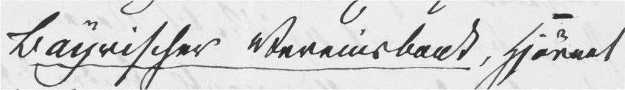Bayrischer Vereinsbank, Hjørnet
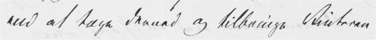end at tage derned og tilbringe Vinteren
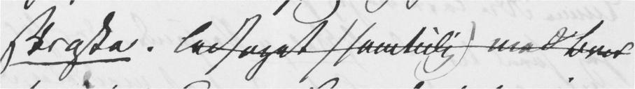strasse. ledsaget (samtidig) med Brev
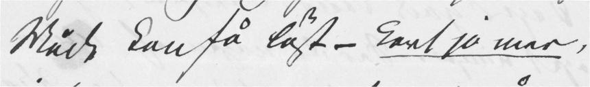Måde kan få løst – kort jo mer,
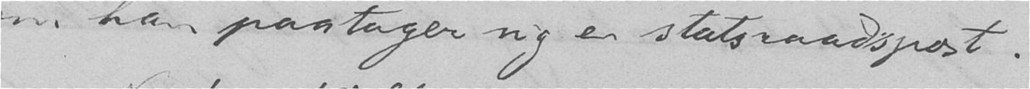om han paatager sig en statsraadspost.
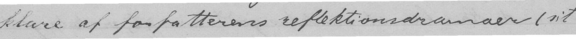klare af forfatterens reflektionsdramaer (sit
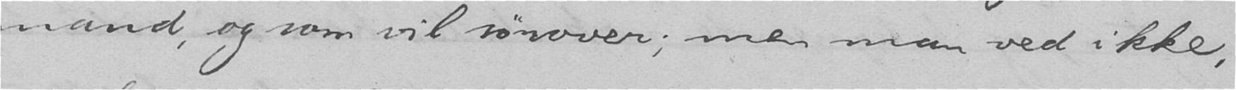mand, og som vil sørover; men man ved ikke,
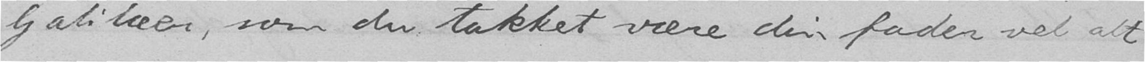Galilæer, som du takket være din fader vel alt
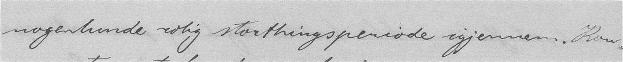nogenlunde rolig storthingsperiode igjennem. Ron-
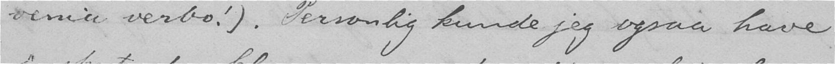venia verbo!). Personlig kunde jeg ogsaa have
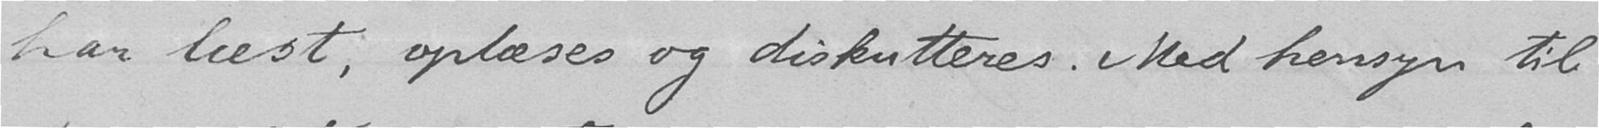har læst, oplæses og diskutteres. Med hensyn til
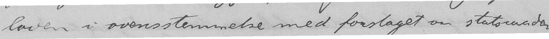loven i ovensstemmelse med forslaget om statsraade-
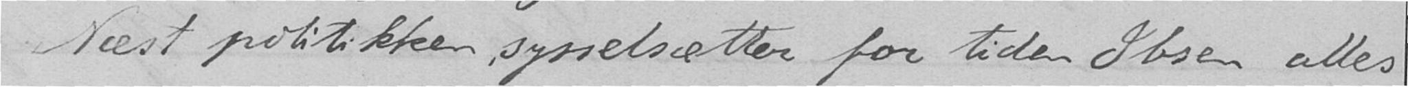Næst politikken sysselsætter for tiden Ibsen alles
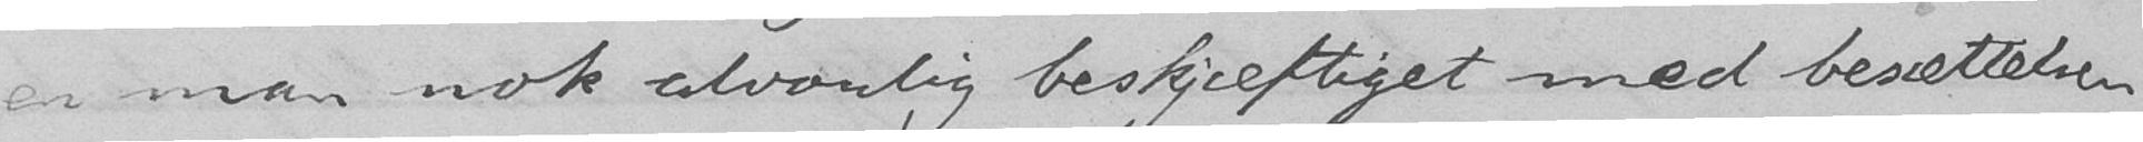er man nok alvorlig beskjæftiget med besættelsen
